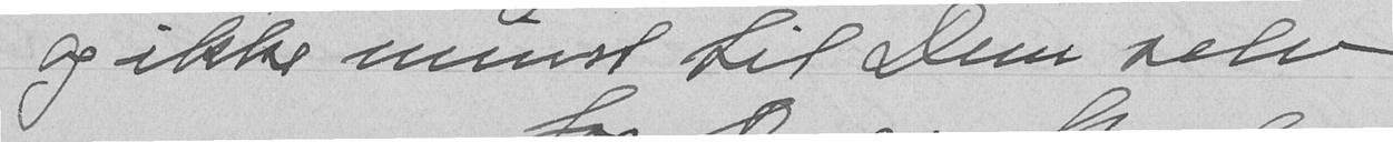og ikke minst til Dem selv
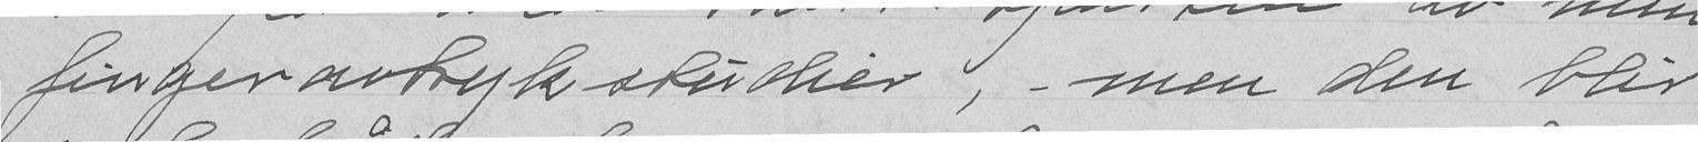fingeravtrykstudier, - men den blir
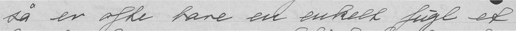så er ofte bare en enkelt fugl et
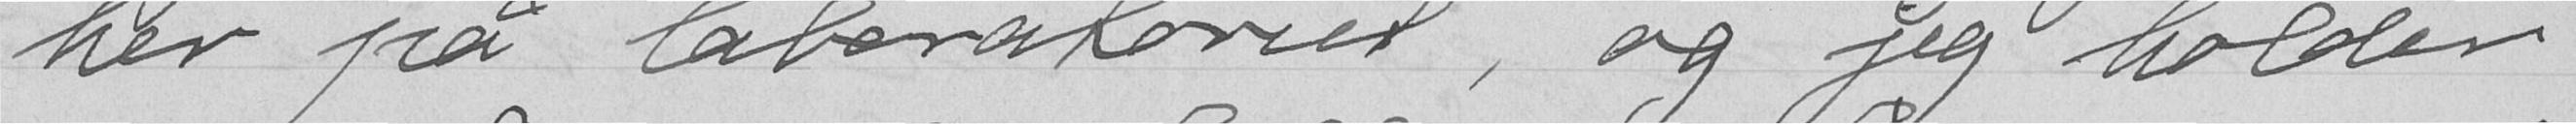her på laboratoriet og jeg holder
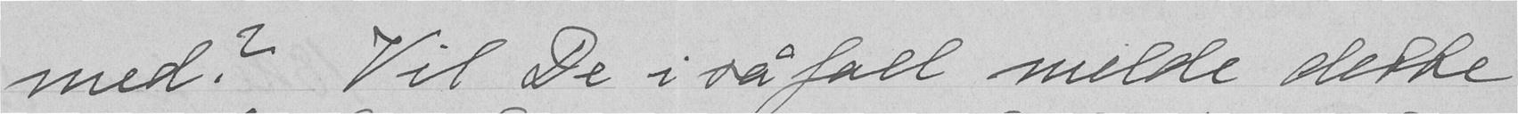med? Vil De i såfall melde dette
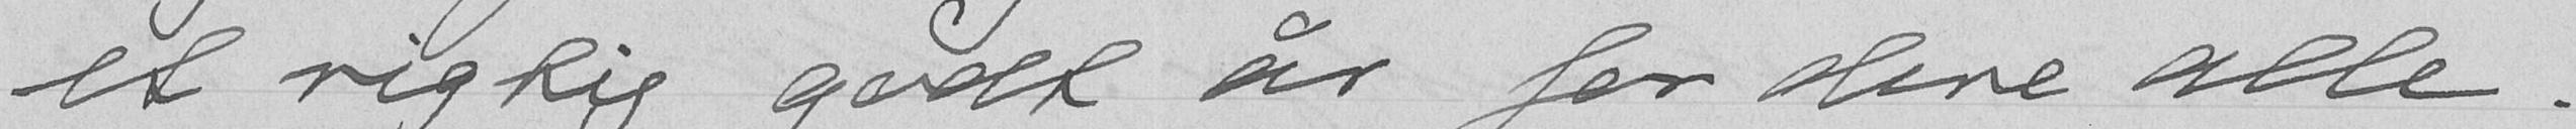et rigtig godt år for dere alle.
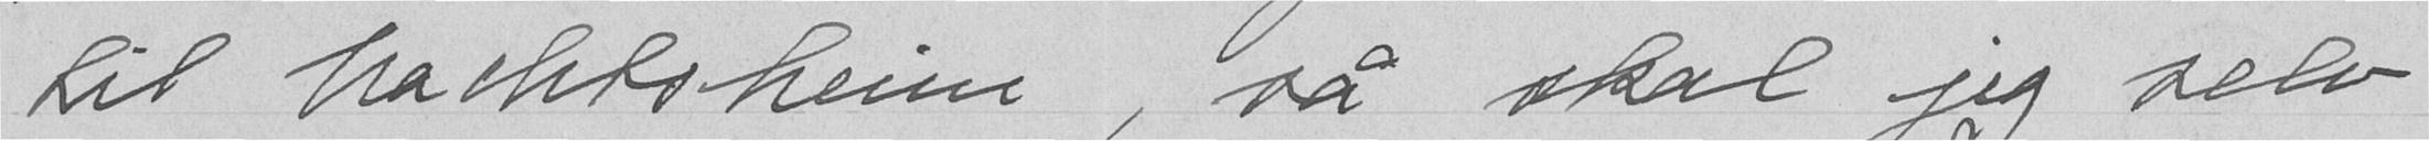til Nachtsheim, så skal jeg selv
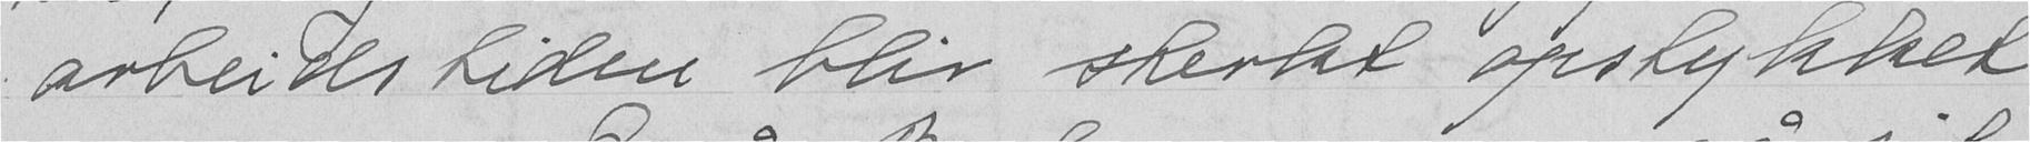arbeidstiden blir sterkt opstykket
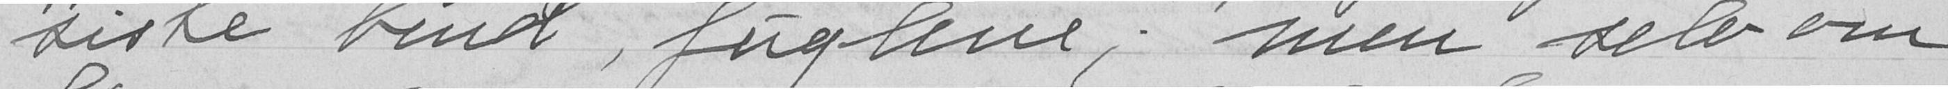siste bind, fuglene, men selv om
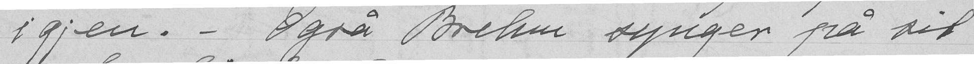igjen. - Også Brelin synger på sit
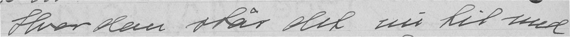Hvordan står det nu til med
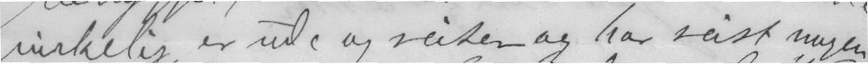virkelig er ude og reiser og har reist nogen
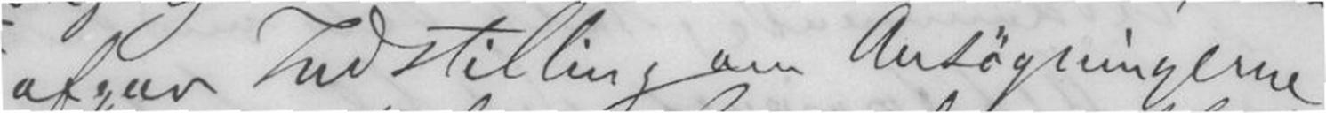afgav Indstillingen om Ansøgningerne
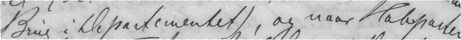Brug i Departementet), og naar Halvparten
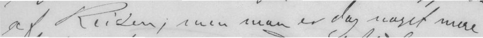af Reisen; men man er dog noget mere
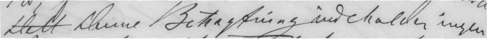Dett Denne Betragtning indeholder ingen
